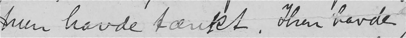hun havde tænkt. Hun havde
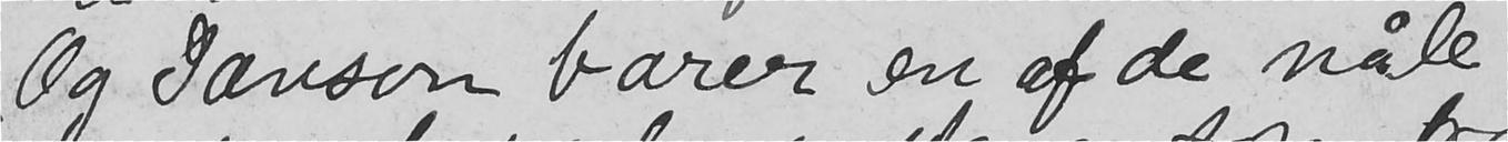Og Janson bærer en af de nåle
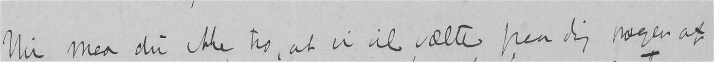Nu maa du ikke tro, at vi vil vælte paa dig nogen af
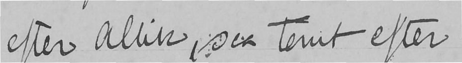efter Allik, saa tomt efter
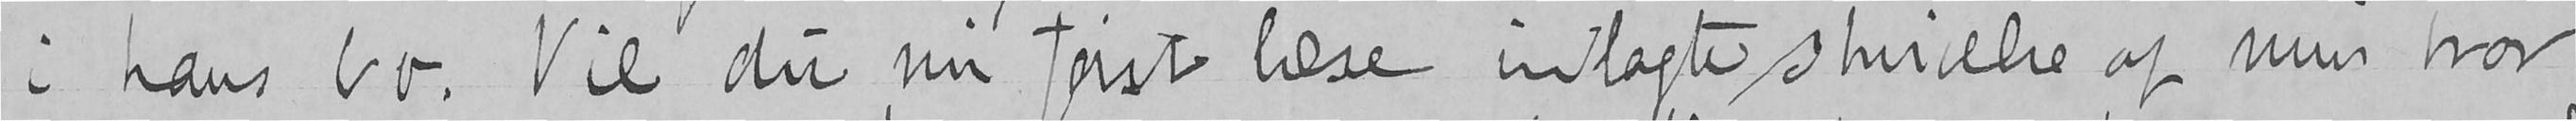i hans bo. Vil du nu først læse indlagte skrivelse af min bror
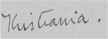Kristiania.
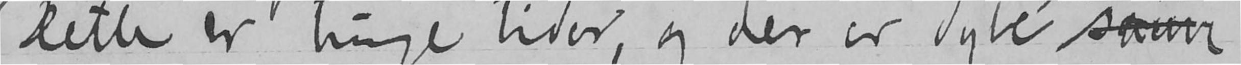Dette er tunge tider, og der er dybe savn
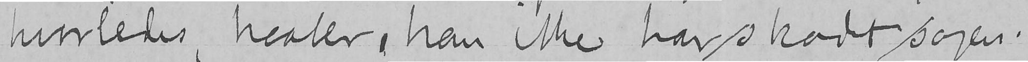hvorledes, haaber, han ikke har skadet sagen.
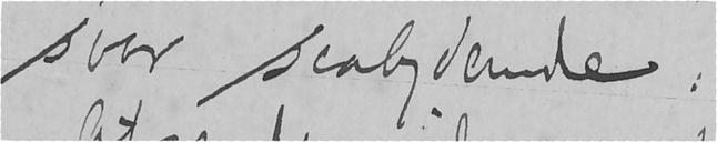svar saalydende:
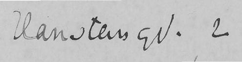Hanstens gd. 2
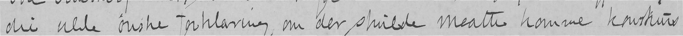du vilde ønske forklaring, om der skulde maatte komme konkurs
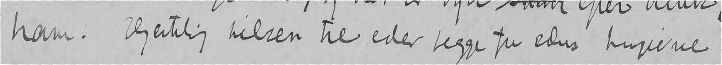ham. Hjertelig hilsen til eder begge fra eders hengivne
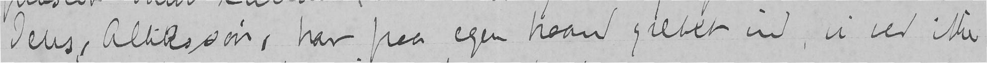Jens, Alliks søn, har paa egen haand grebet ind, vi ved ikke
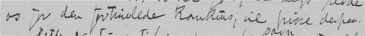os for den fortvivlede konkurs, vil prøve derpaa.
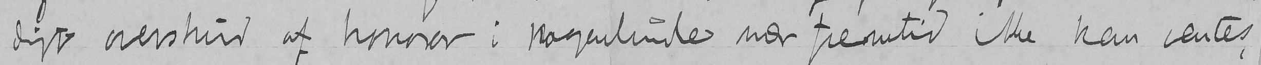digt overskud af honorar i nogenlunde nær fremtid ikke kan ventes,
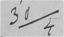30/4
